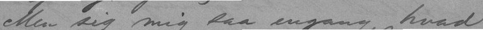Men sig mig saa engang, hvad
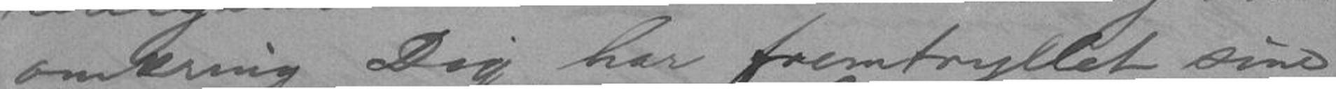omkring Dig har fremtryllet sine
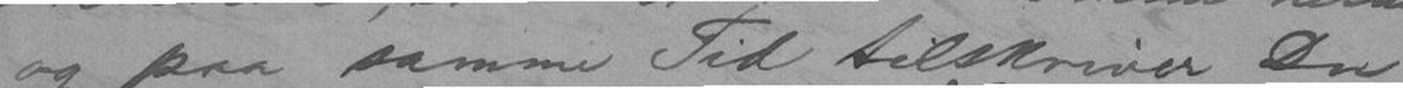og paa samme Tid tilskriver Du
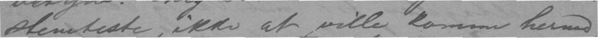stemteste, ikke at ville komme herned,
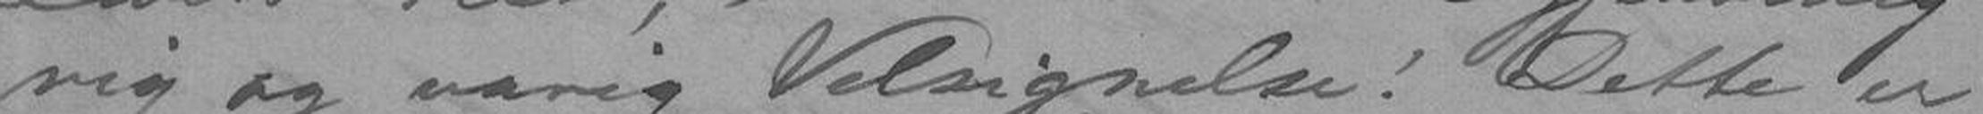rig og varig Velsignelse! Dette er
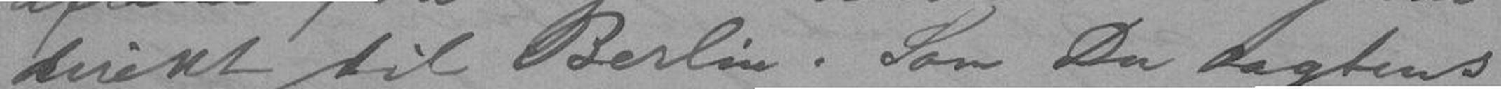direkt til Berlin. Som Du sagtens
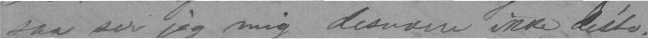saa ser jeg mig desværre ikke desto-
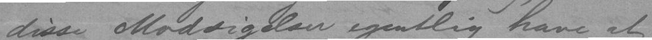disse Modsigelser egentlig have at
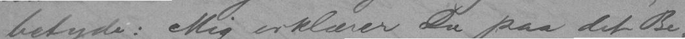betyde: Mig erklærer Du paa det Be-
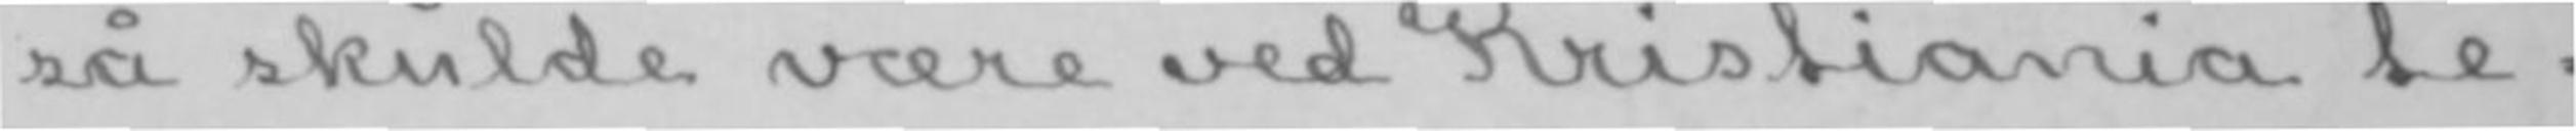så skulde være ved Kristiania te-
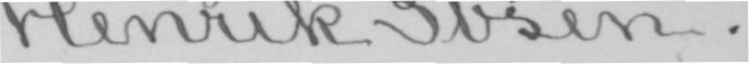Henrik Ibsen.
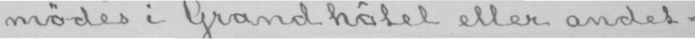mødes i Grand hôtel eller andet-
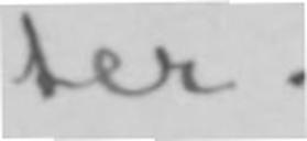ter.
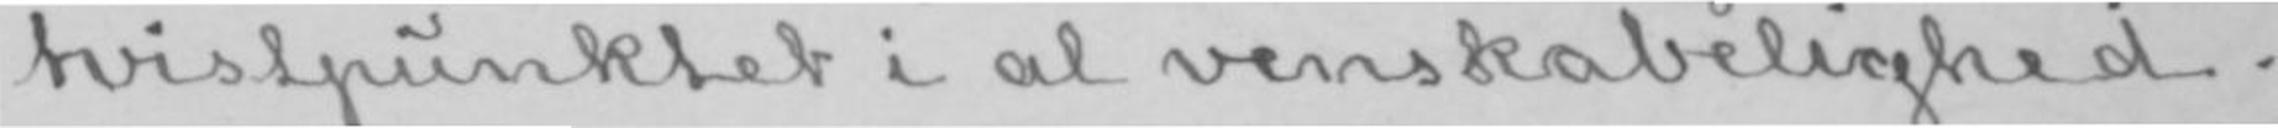tvistpunktet i al venskabelighed.
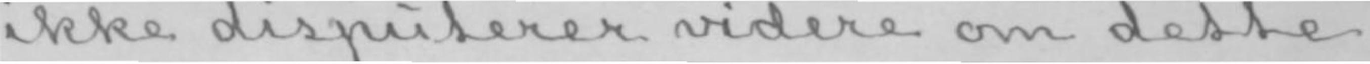ikke disputerer videre om dette
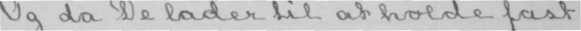Og da De lader til at holde fast
